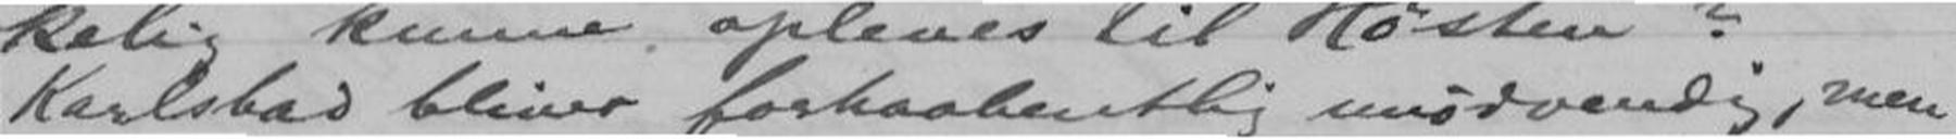Karlsbad bliver forhaabentlig unødvendig, men
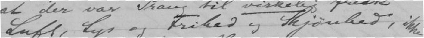Luft, Lys og Frihed og Skjønhed, ikke
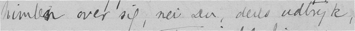himlen over sig, nei Du, deres udtryk,
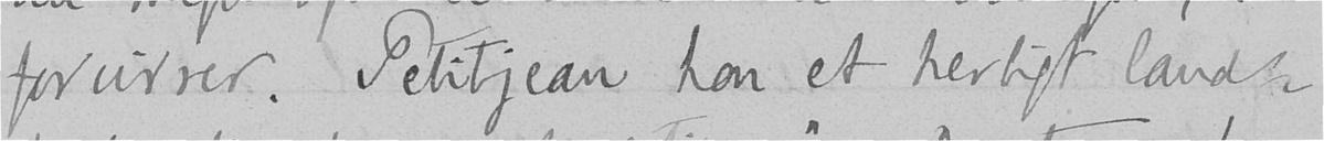forvirrer. Petitjean har et herligt land-
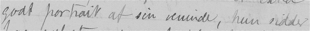godt portrait af sin veninde, hun sidder
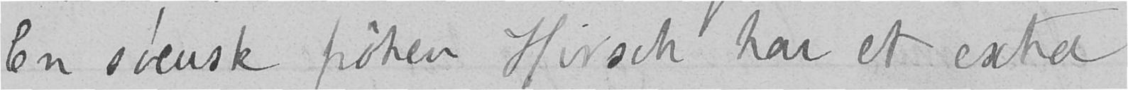En svensk frøken Hirsch har et extra
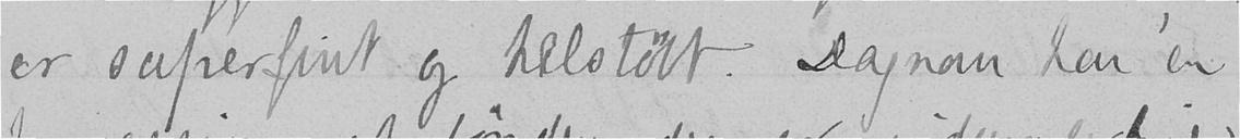er superfint og helstøbt. Dagnan har en
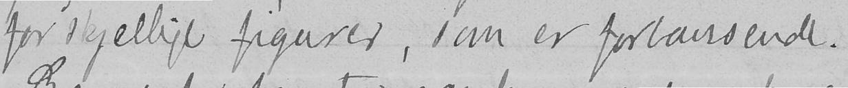forskjellige figurer, som er forbausende.
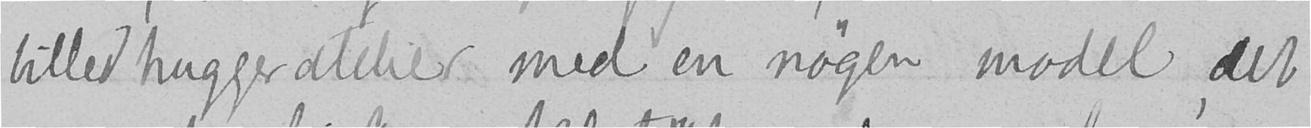billedhuggeratelier med en nøgen model, det
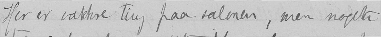Her er vakkre ting paa salonen, men noget
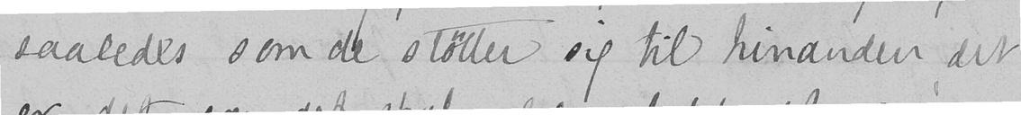saaledes som de støtter sig til hinanden, det
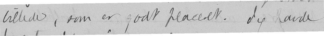billede, som er godt placeret. Jeg havde
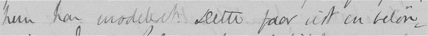hun har modeleret. Dette faar vist en beløn-
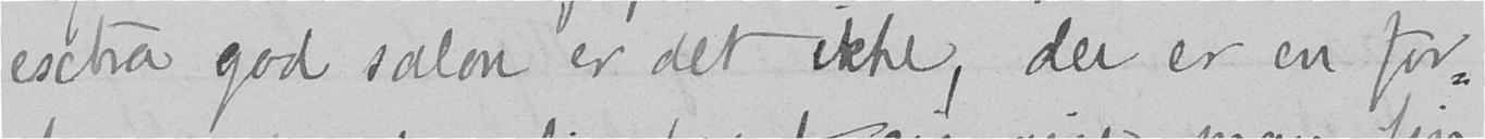extra god salon er det ikke, der er en for-
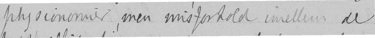physionomier, men misforhold imellem de
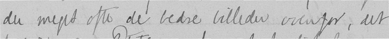der meget ofte de bedre billeder ovenfor, det
